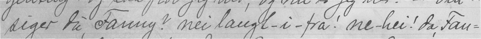siger du, Fanny? nei langt- i-fra! ne-hei! da, Fan-
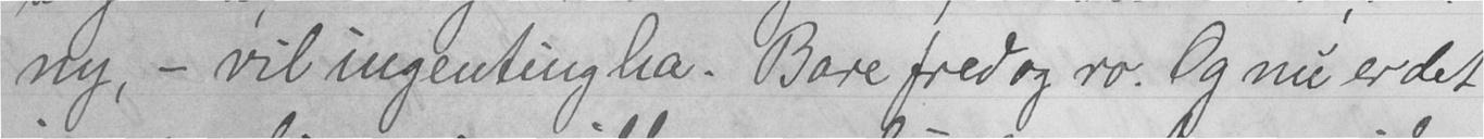ny, - vil ingenting ha. Bare fred og ro. Og nu er det
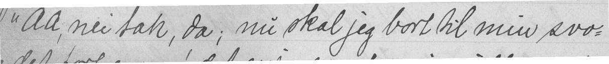"Aa, nei tak, da; nu skal jeg bort til min svo-
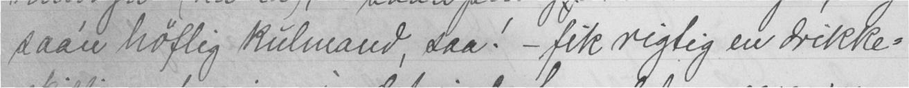saa'n høflig kulmand, saa! - fik rigtig en drikke-
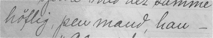høflig, pen mand, han -
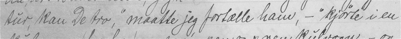tur, kan De tro," maatte jeg fortælle ham, - "kjørte i en
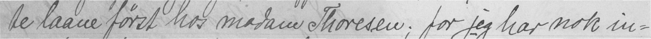te laane først hos madam Thoresen; for jeg har nok in-
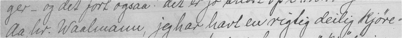Aa, hr. Waalmann, jeg har havt en rigtig deilig kjøre-
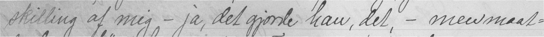skilling af mig - ja, det gjorde han, det, - men maat-
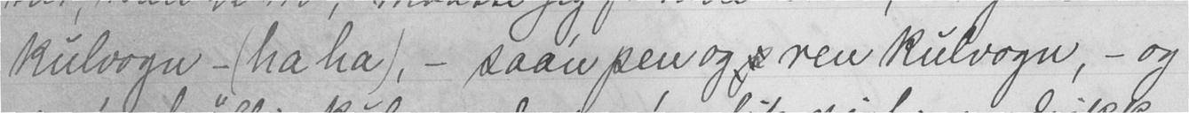kulvogn - (ha ha), - saa'n pen og p ren kulvogn, - og
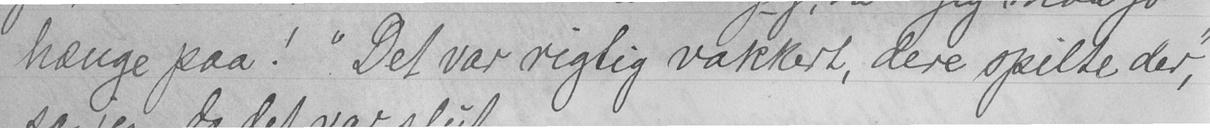hænge paa! "Det var rigtig vakkert, dere spilte der,"
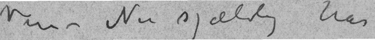Ven – Nu sjælelig har
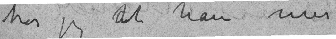tror jeg at han mer
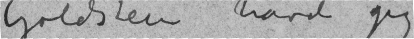Goldstein havde jeg
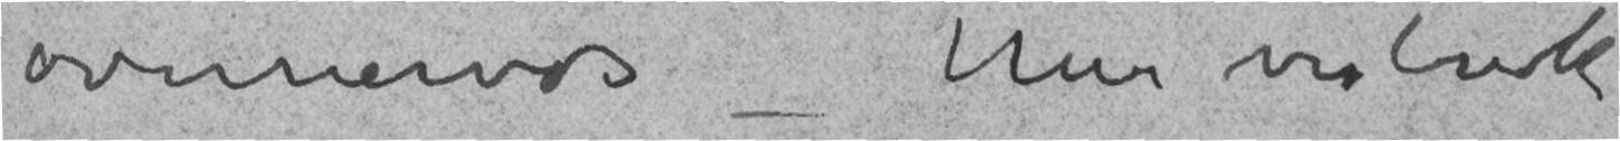overnervøs – Men vistnok
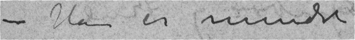– Han er mindst
